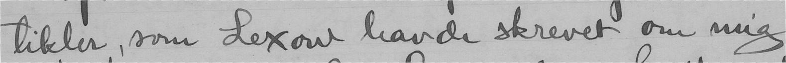tikler, som Lexow havde skrevet om mig
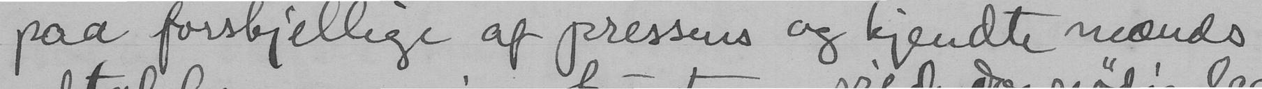paa forskjellige af pressens og kjendte mænds
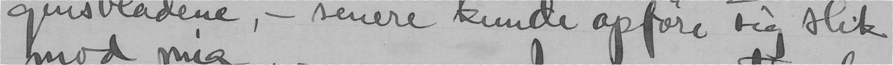gensbladene, - senere kunde opføre sig slik
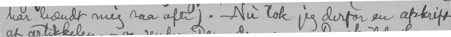har hændt mig saa ofte). Nu tok jeg derfor en afskrift
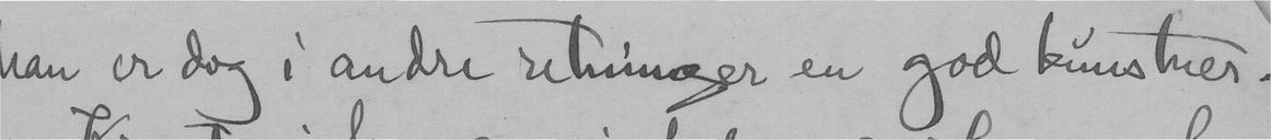han er dog i andre retninger en god kunstner.
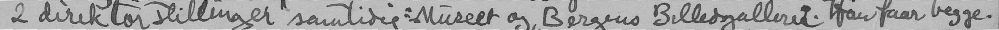2 direktørstillinger samtidig: Museet og "Bergens Billedgalleri". Han faar begge.
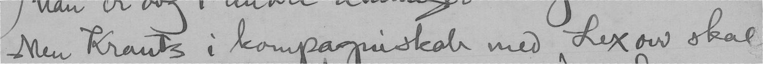Men Krantz i kompagniskab med Lexow skal
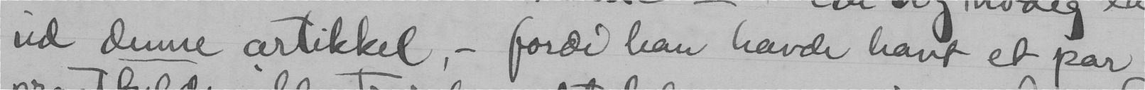ud denne artikkel, - fordi han havde havt et par
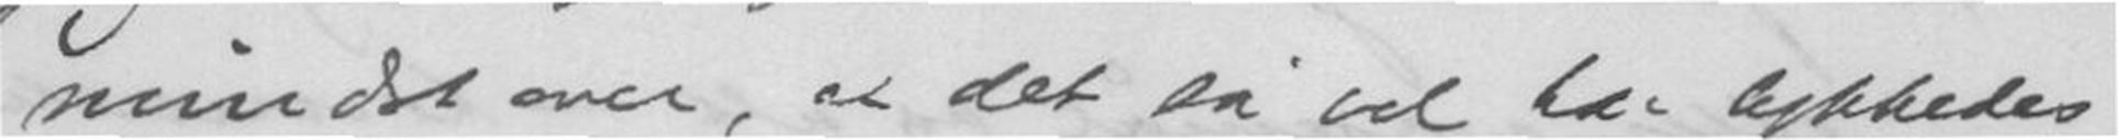mindst over, at det så vel har lykkedes
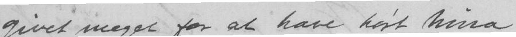givet meget for at have hørt Nina
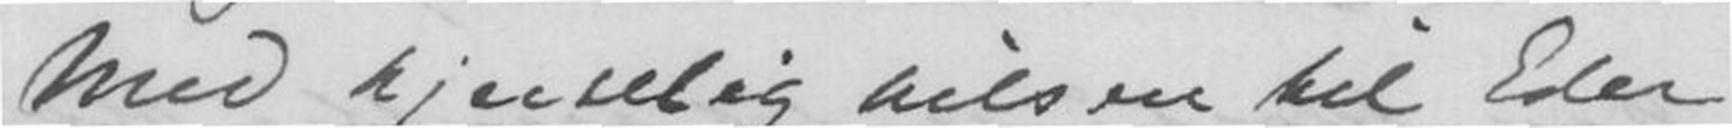Med hjertelig hilsen til Eder
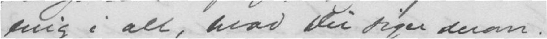enig i alt, hvad Du siger derom.
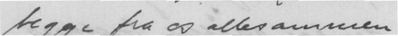begge fra os allesammen
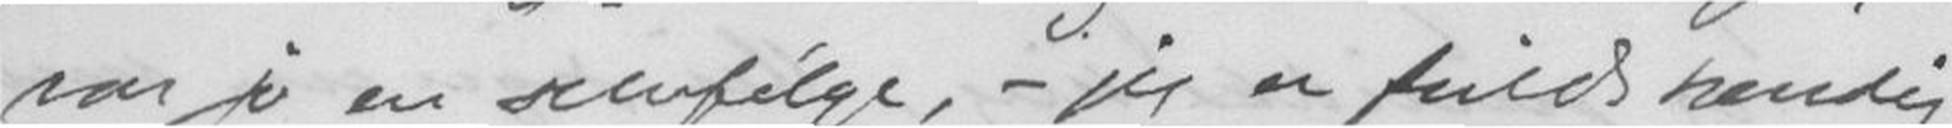var jo en selvfølge, - jeg er fuldstændig
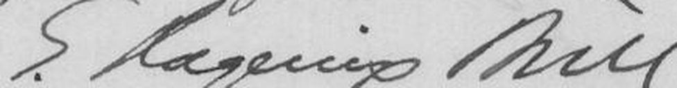E. Hagerup Bull.
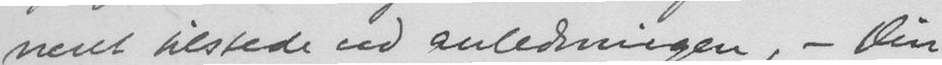veret tilstedet ved anledningen, - Din
